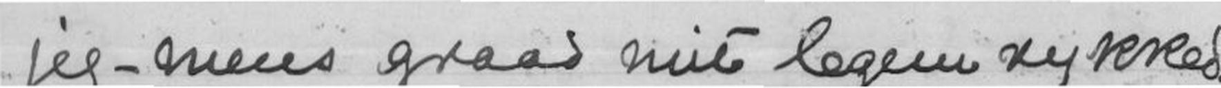jeg - mens graad mit legem rykked
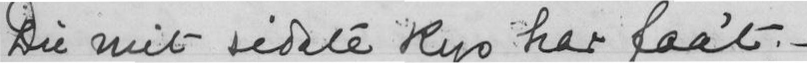Du mit sidste kys har faat; -
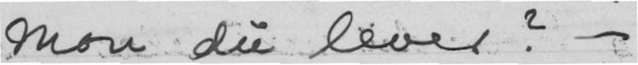Mon du lever? -
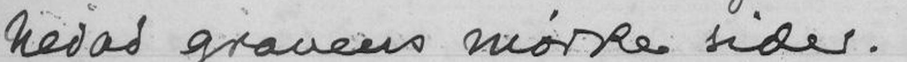nedad gravens mørke sider.
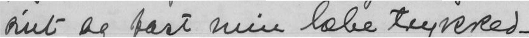But og fast min læbe trykked -
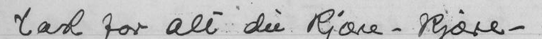- tak for alt, du kjære - kjære -
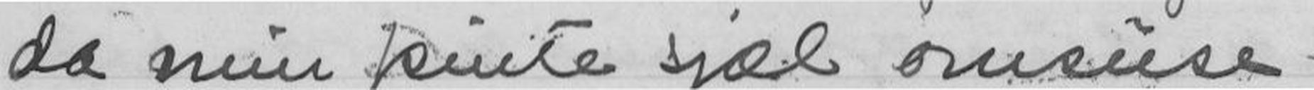da min pinte sjæl omsuse -
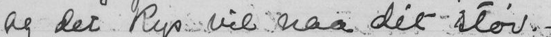og det kys vil naa dit støv. -
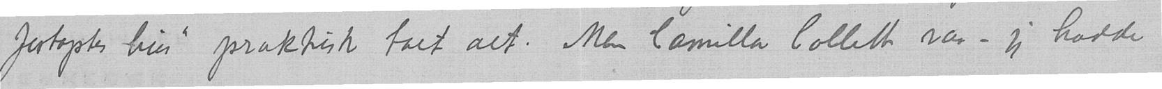fortaptes hus" praktisk talt alt. Men Camilla Colletts var – jeg hadde
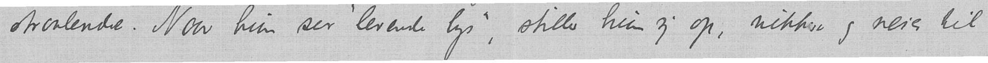straalende. Naar hun ser "levende lys", stiller hun sig op, nikker og neier til
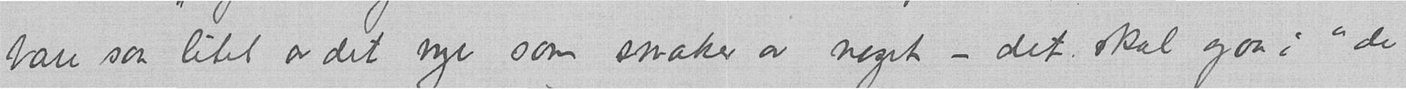bare saa litet av det nye som smaker av noget – det skal gaa i "de
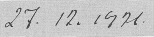27. 12.1926.
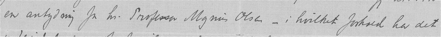en antydning fra hr. Professor Magnus Olsen – i hvilket forhold har det
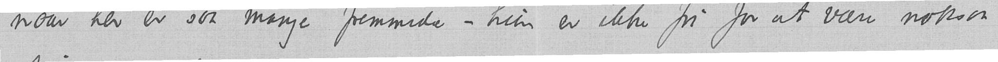naar her er saa mange fremmede – hun er ikke fri for at være noksaa
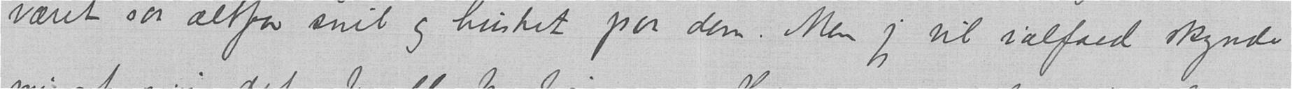været saa altfor snil og husket paa dem. Men jeg vil ialfald skynde
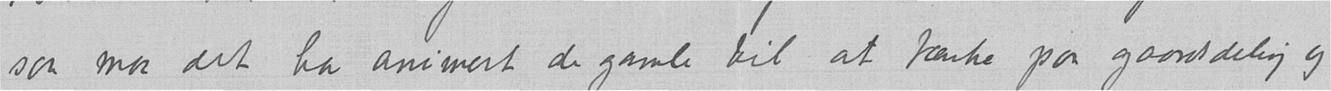saa maa det ha animert de gamle til at tænke paa gaardsdeling og
